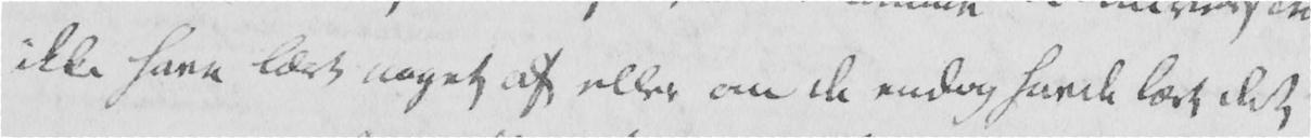ikke have lært noget af eller om de endog havde lært det
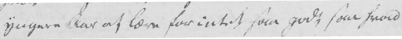yngere var at lære for intet saa godt som hvad
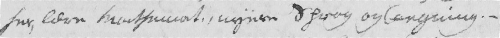her, lære Mathematik, nyere Sprog og Tegning. -
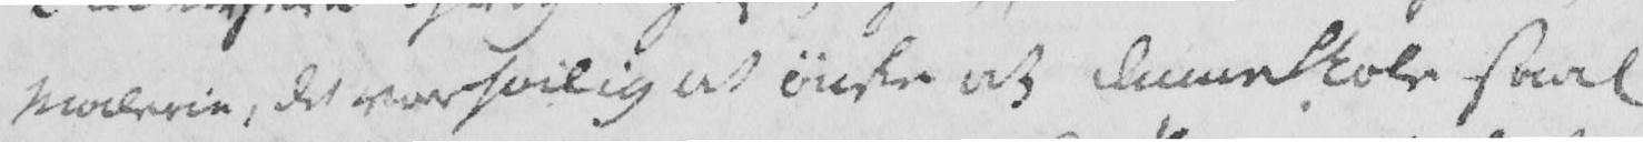malerie, det var høilig at ønske at denne Skole saal
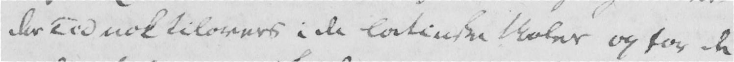der Tid nok tilovers i de latinske Skoler og for de
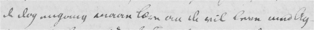de dog engang maae lære om de vil leve med Ag-
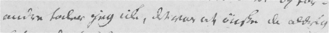andre taler jeg icke, det var at ønske de aldrig
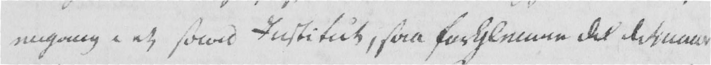engang i et saad Institut, saa forglemme de det naar
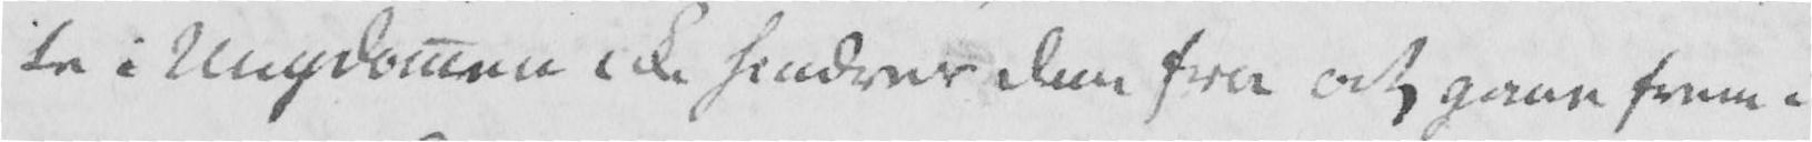te i Ungdomen icke hindrer dem fra at gaae frem i
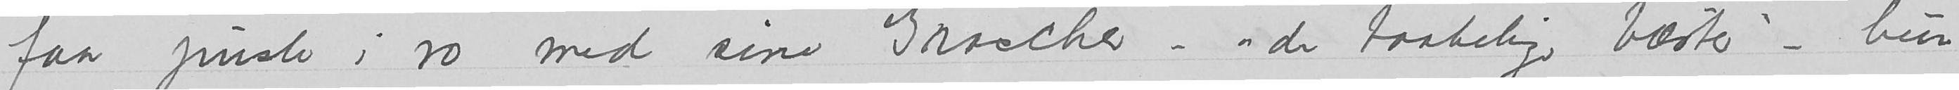faa pusle i ro med sine Graccher – «de taabelige bæster» – hun
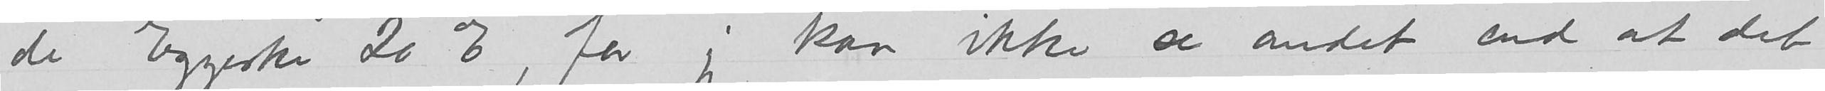de Eggeske 20%, for jeg kan ikke se andet end at det
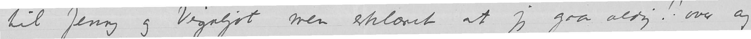til Jenny og Vigaljort men erklæret at jeg gaar aldrig!! over og
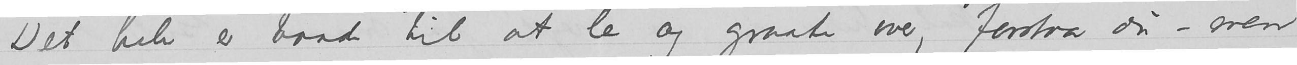Det hele er baade til at le og graate over, forstaar du – men
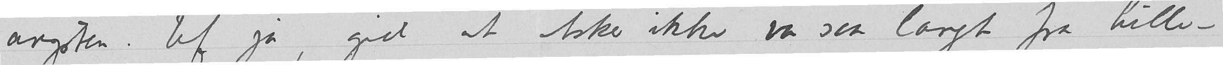den angsten. Uf ja, gid at Asker ikke var saa langt fra Lille-
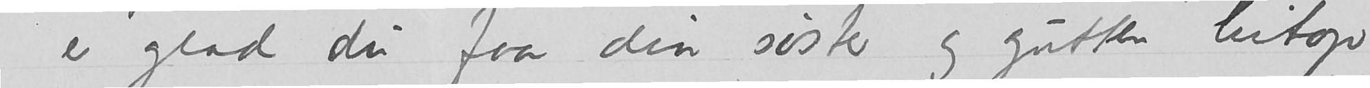Jeg er glad du faar din søster og gutten hitop
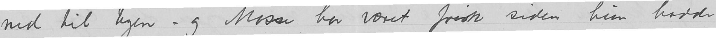ned til byen – og Mosse har været frisk siden hun hadde
